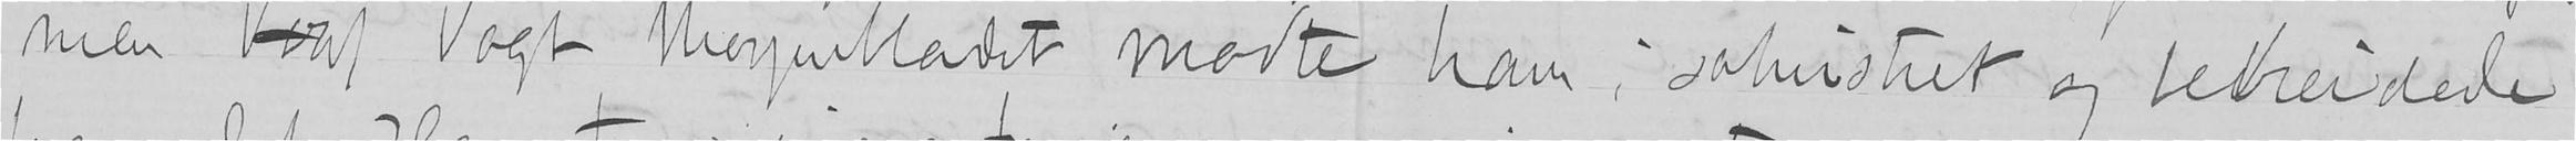men Vont Vogt Morgenbladet mødte ham i sakristiet og bebreidede
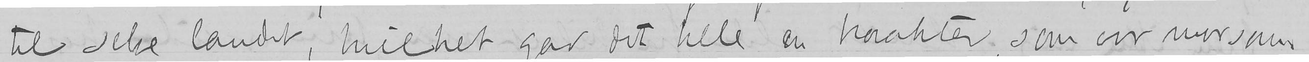til selve landet, hvilket gav det hele en karakter, som var morsom
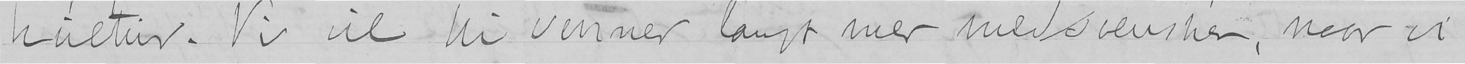kultur. Vi vil bli venner langt mer medsvensker, naar vi
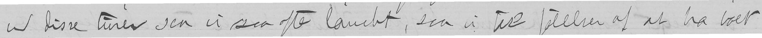ved disse turer saa vi saa ofte landet, saa vi fik følelsen af at ha boet
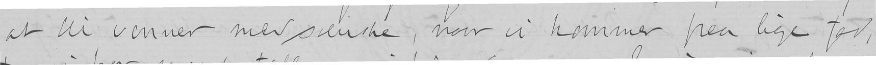at bli venner med svenske, naar vi kommer paa lige fod,
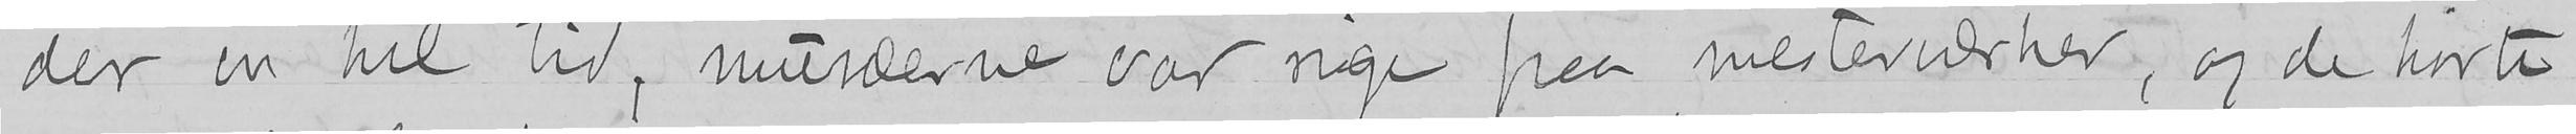der en hel tid, musæerne var rige paa mesterværker, og de hørte
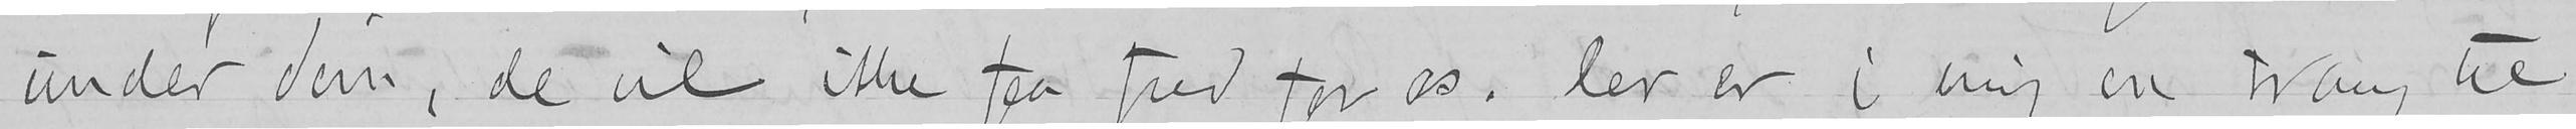under dem, de vil ikke faa fred for os. Der er i mig en trang til
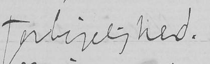forligelighed.
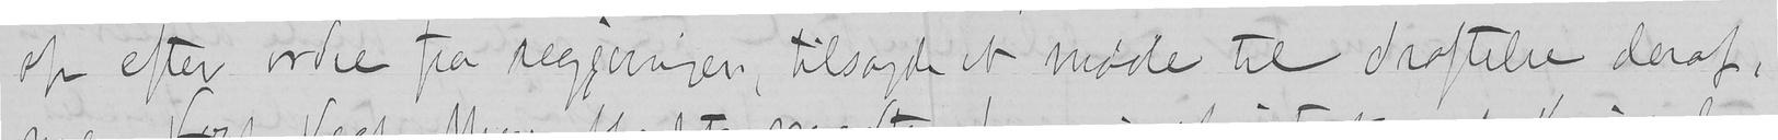op efter ordre fra regjeringen, tilsagde et møde til drøftelse deraf,
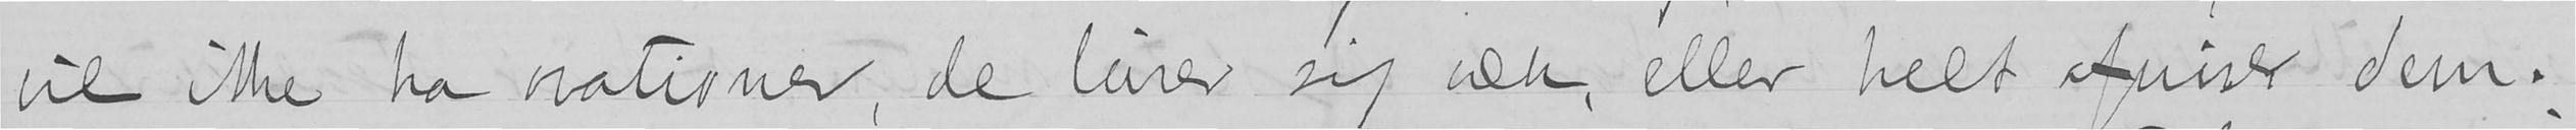vil ikke ha ovationer, de lurer sig væk, eller helt afviser dem.
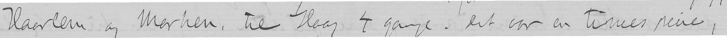Haarlem og Marhen, til Haag 4 gange. Det var en times reise,
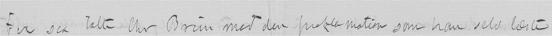Fra saa talte Chr Brun med den proklamation som han selv læste
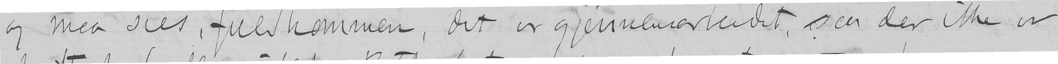og maa sies, fuldkommen, det er gjennemarbeidet, saa der ikke er
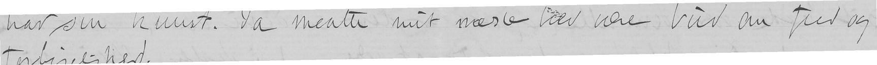har sin kunst. Ja maatte mit næste brev være bud om fred og
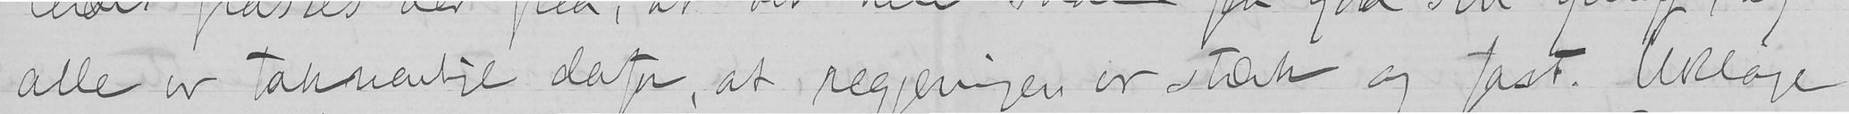alle er taknemlige derfor, at regjeringen er stærk og fast. Ukloge
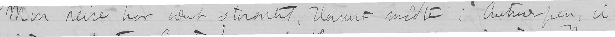Min reise har vært storartet, Harriet mødte i Antwerpen, vi
